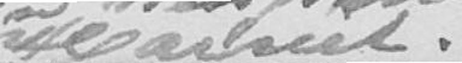Harriet.
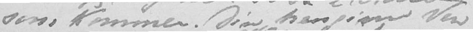som Kommer. Din hengivne Ven
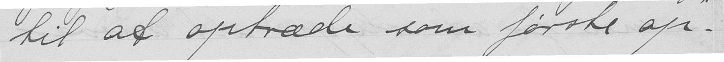til at optræde som første op-
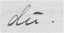du.
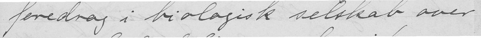foredrag i biologisk selskab over
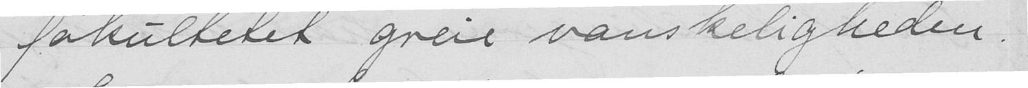fakultetet greie vanskeligheden.
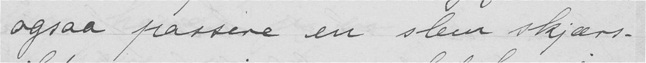ogsaa passere en slem skjærs-
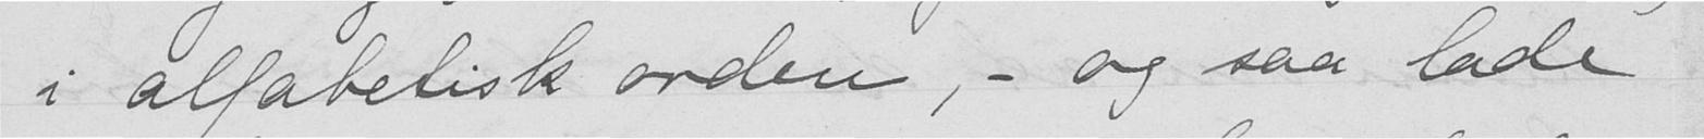i alfabetisk orden, - og saa lade
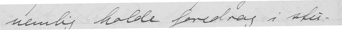nemlig holde foredrag i stu-
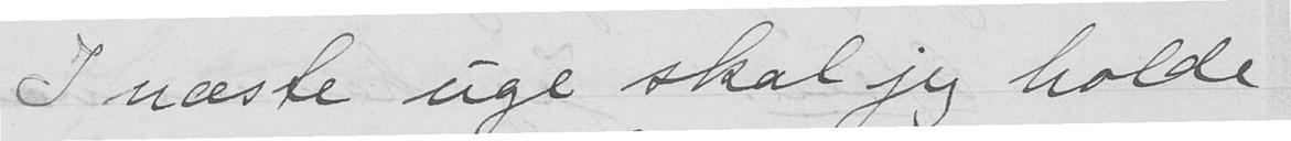I næste uge skal jeg holde
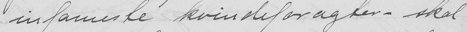infameste kvindeforagter - skal
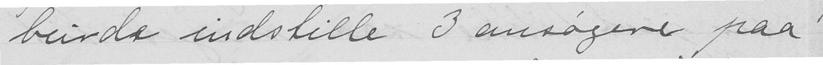burde indstille 3 ansøgere paa
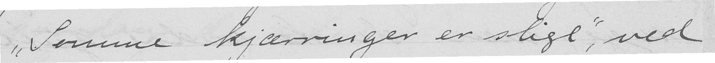"Somme kjærringer er slige", ved
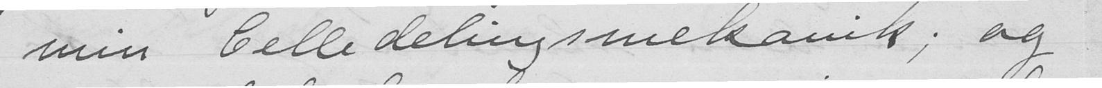min Celledelingsmekanik; og
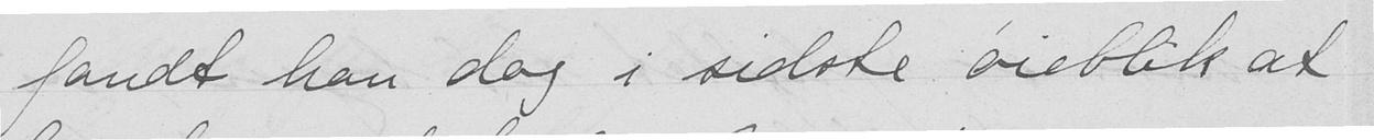fandt han dog i sidste øieblik at
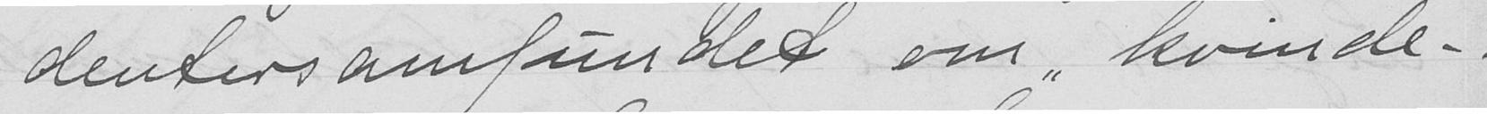dentersamfundet om "kvinde-
